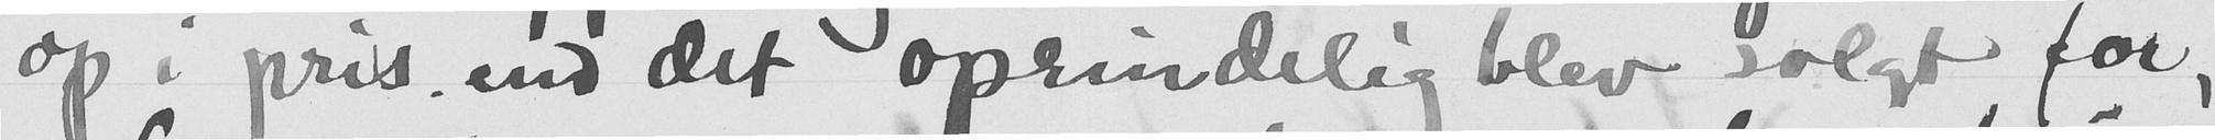op i pris end det oprindelig blev solgt for,
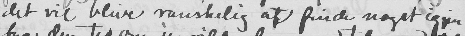det vil blive vanskelig at finde noget igjen
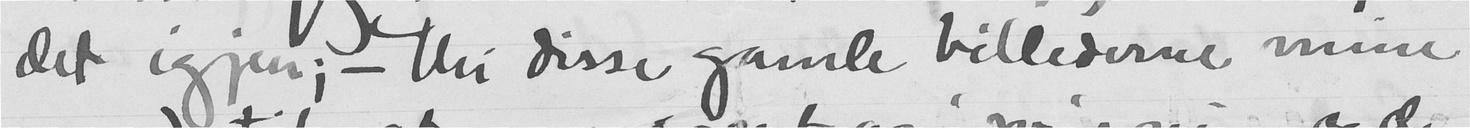det igjen; - thi disse gamle billederne mine
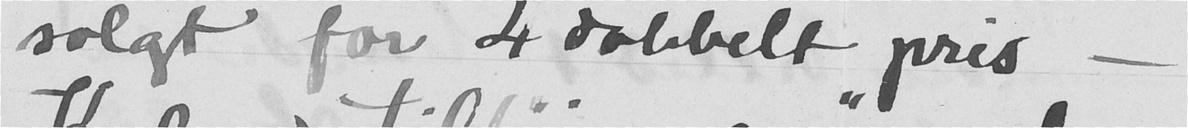solgt for 4 dobbelt pris -
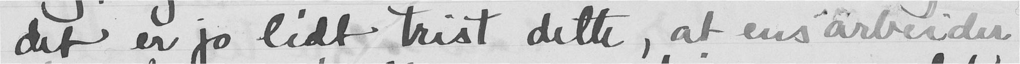det er jo lidt - trist dette, at ens arbeider
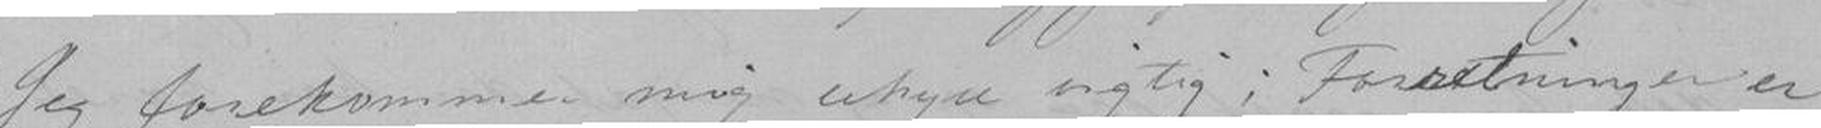Jeg forekommer mig uhyre vigtig; Forretninger er
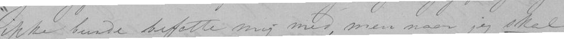ikke burde befatte mig med, men naar jeg skal
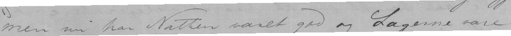men nu har Natten været god og Lægerne være
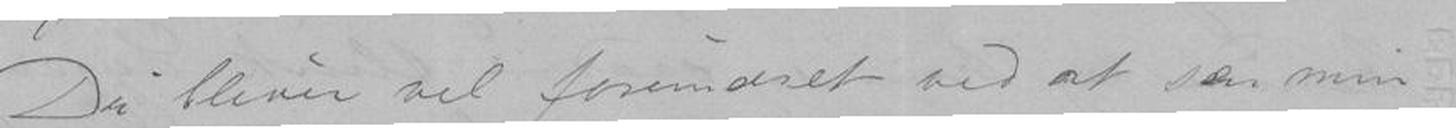Du bliver vel forundret ved at se min
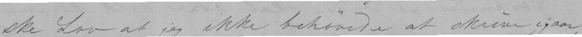ske Lov at jeg ikke behøvede at skrive igaar,
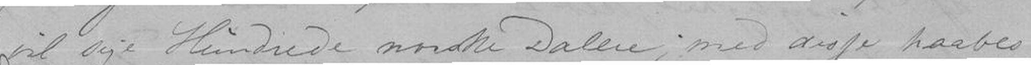vil sige Hundrede norske Dalere; med disse haabes
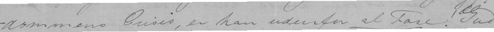dommens Cusis, er han udenfor al Fare. Gud
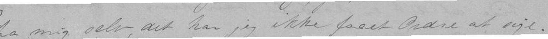fra mig selv, det har jeg ikke faaet Ordre at sige.
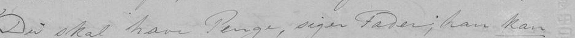Du skal have Penge, siger Fader; han kan
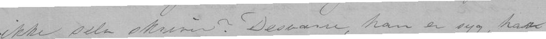ikke selv skriver? Desværre, han er syg, har
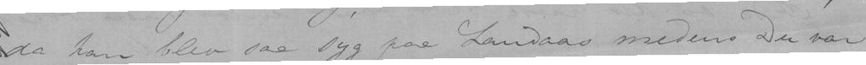da han blev saa syg paa Landaas medens Du var
